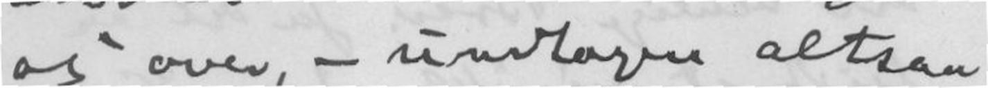os over, - undtagen altsaa
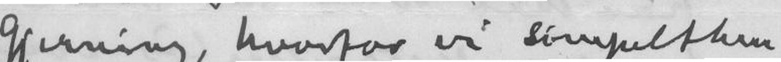Gjerning, hvorfor vi simpelthen
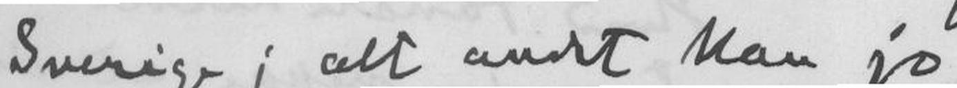Sverige; alt andet kan jo
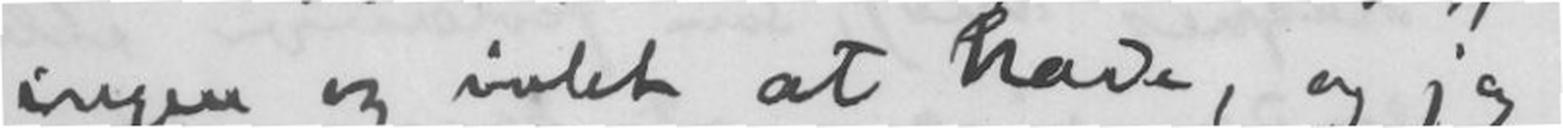ingen og intet at hade, og jeg
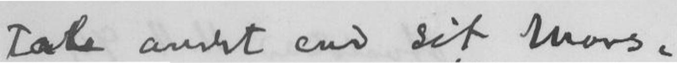Tale andet end sit Mors-
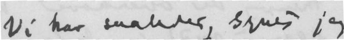Vi har saaledes, synes jeg
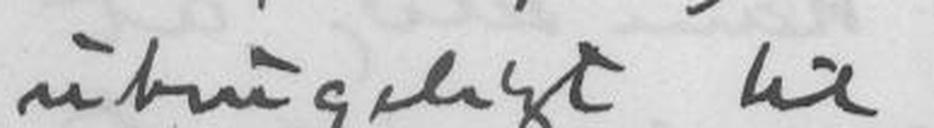ubrugeligt til
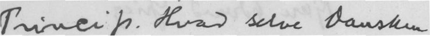Princip. Hvad selve Dansken
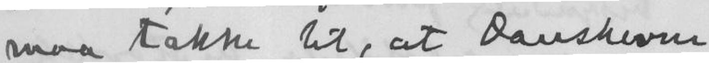maa takke til, at Danskerne
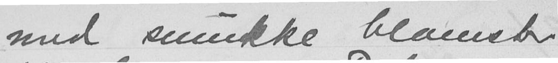med smukke blomster
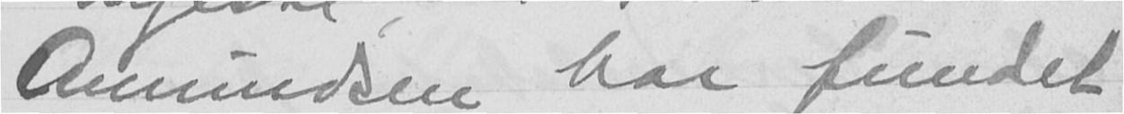Amundsen har fundet
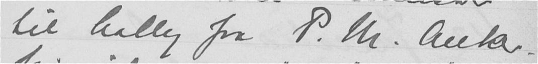til Cally fra P. M. Anker.
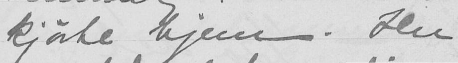kjørte hjem. Her
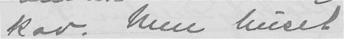kav. Men huset
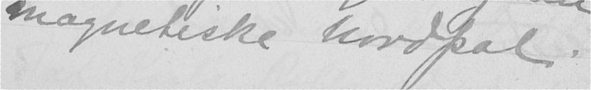magnetiske Nordpol.
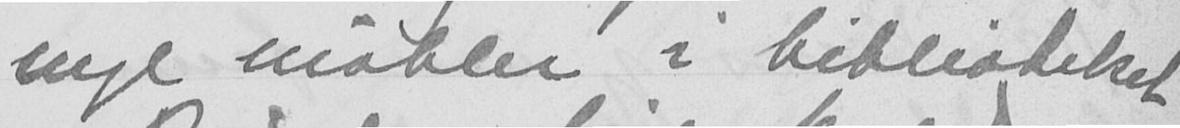nye møbler i biblioteket
